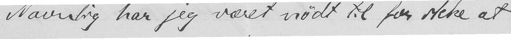Navnlig har jeg været nødt til for ikke at
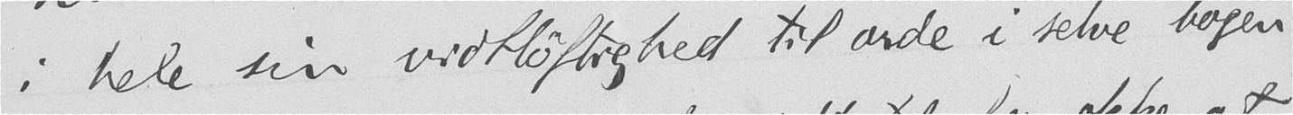i hele sin vidtløftighed til orde i selve bogen.
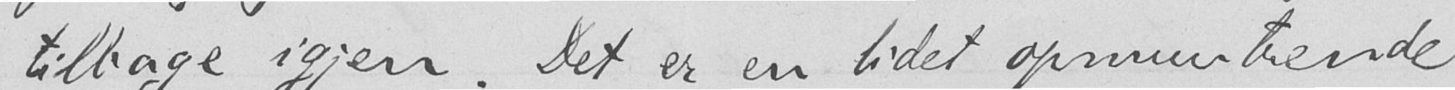tilbage igjen. Det er en lidet opmuntrende
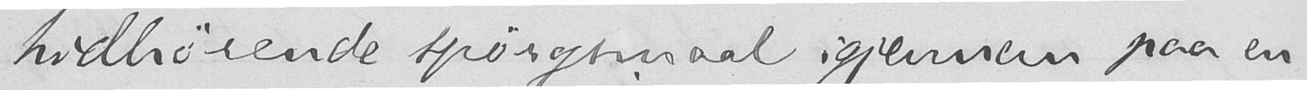hidhørende spørgsmaal igjennem paa en
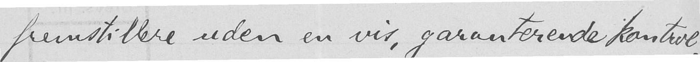fremstillere uden en vis, garanterende kontrol,
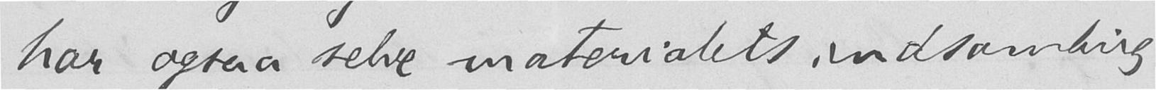har ogsaa selve materialets indsamling
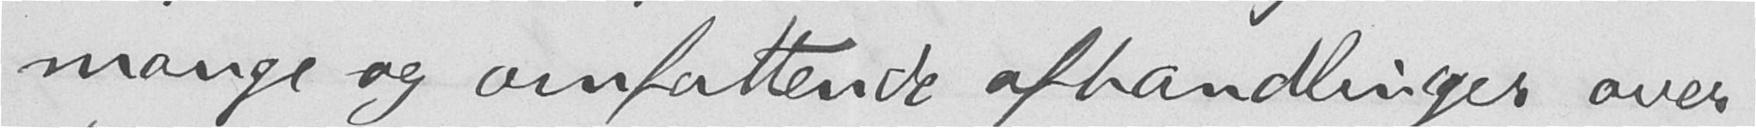mange og omfattende afhandlinger over
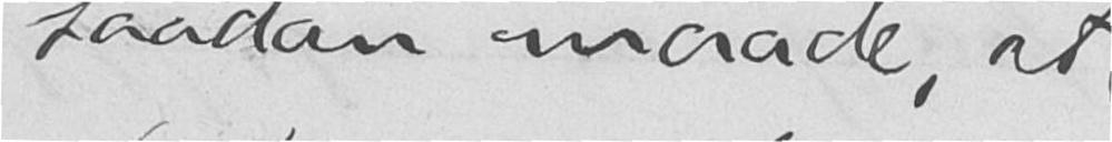saadan maade, at
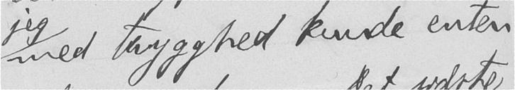med trygghed kunde enten
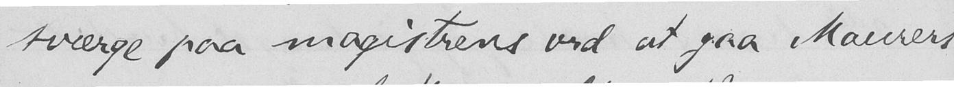sværge paa magistrens ord at gaa Maurers
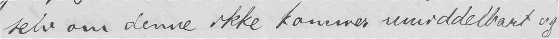selv om denne ikke kommer umiddelbart og
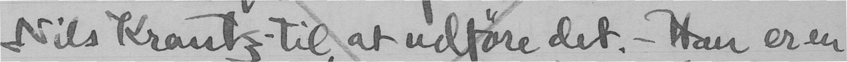Nils Krantz til at udføre det. - Han er en
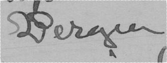Bergen
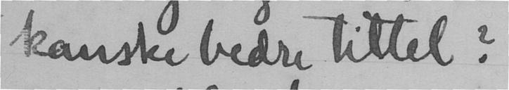kanske bedre tittel?
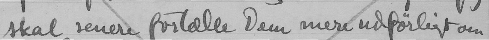skal senere fortælle Dem mere udførligt om
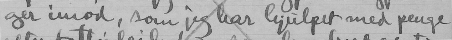ger imod, som jeg har hjulpet med penge
